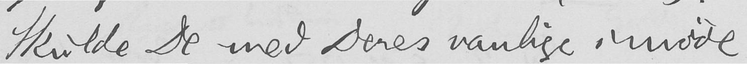Skulde De med Deres vanlige imøde-
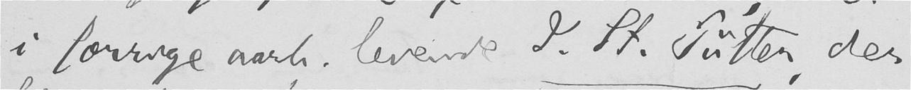i forrige aarh. levende J. St. Pütter, der
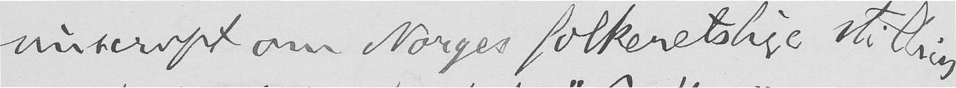nuscript om Norges folkeretslige stilling
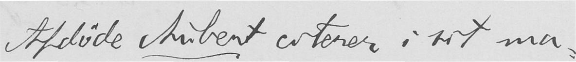Afdøde Aubert citerer i sit ma-
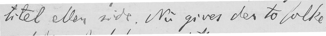titel eller side. Nu gives der to folke-
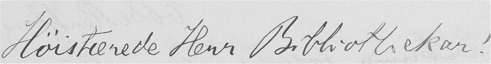Høistærede Herr Bibliothekar!
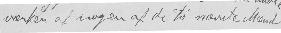værker af nogen af de to nævnte Mænd
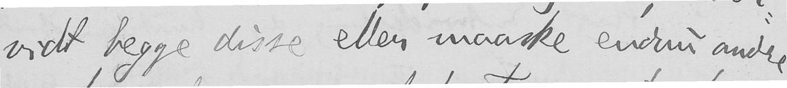vidt begge disse eller maaske endnu andre
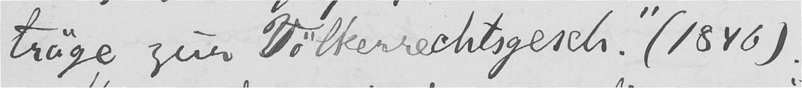träge zur Völkerrechtsgesch." (1846).
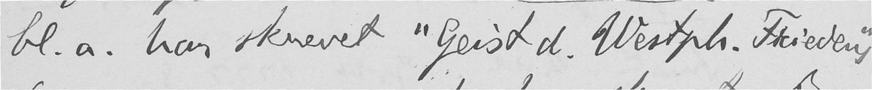bl. a. har skrevet "Geist d. Westph. Friedens"
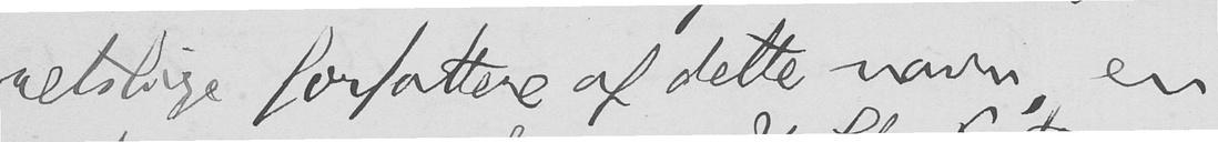retslige forfattere af dette navn, en
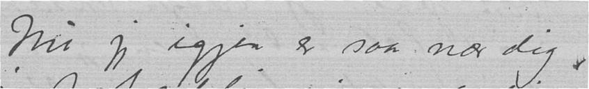Nu jeg igjen er saa nær dig,
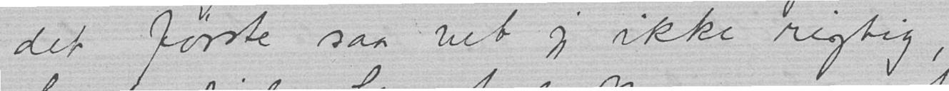det første saa vet jeg ikke rigtig,
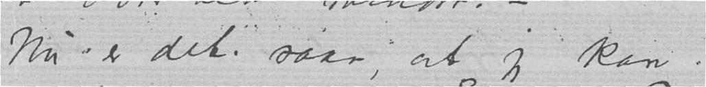Nu er det saan, at jeg kan
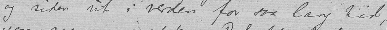og siden ut i verden for saa lang tid,
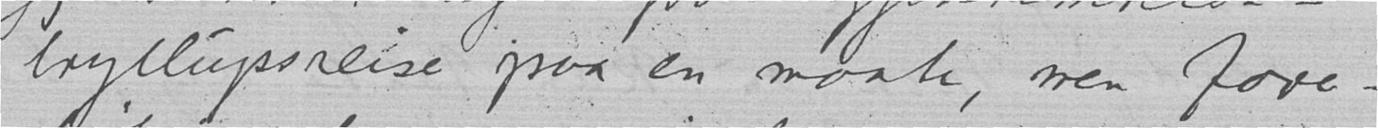bryllupsreise paa en maate, men fore-
